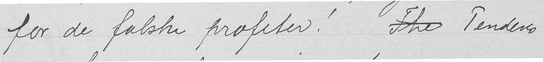for de falske profeter! The Tendens
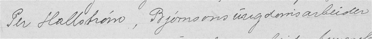Per Hallstrøm, Bjørnsons ungdomsarbeider
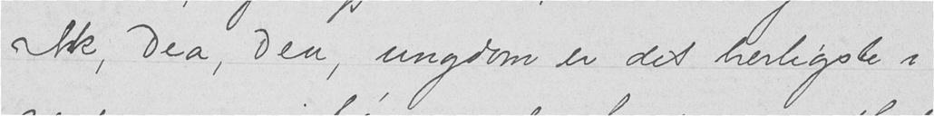Ak, Dea, Dea, ungdom er det herligste i
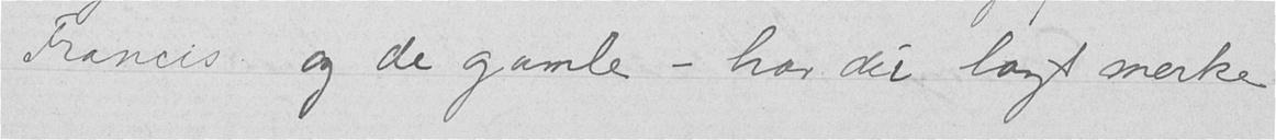Francis og de gamle - har du lagt merke
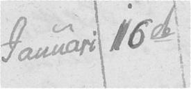Januari 16de
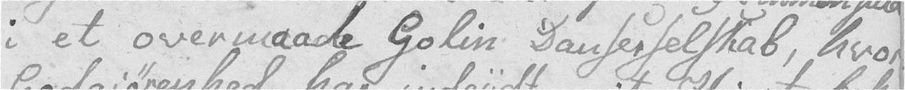i et overmaade Golin Danserselskab, hvor
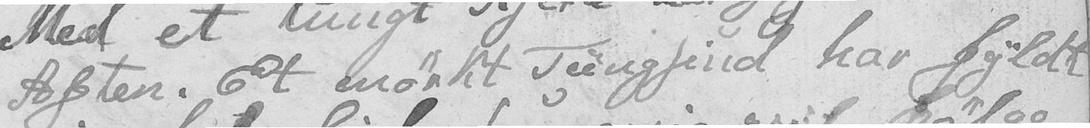Aften. Et mørkt Tungsind har fyldt
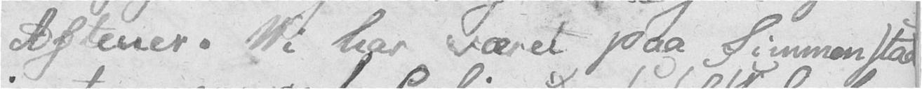Aftener. Vi har været paa Simmenstad
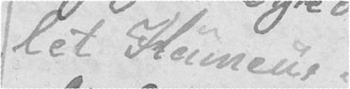let Humeur.
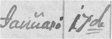Januari 17de
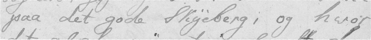paa det gode Skyeberg, og hvor
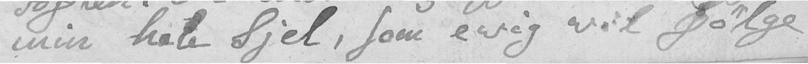min hele Sjel, som evig vil følge
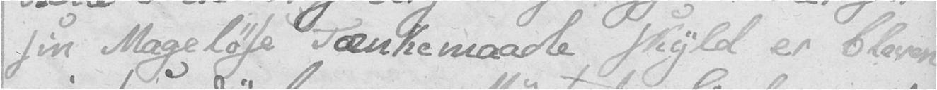sin Mageløse Tænkemaade skyld er bleven
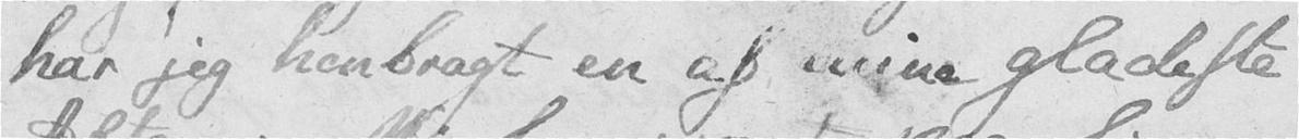har jeg henbragt en af mine gladeste
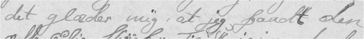det glæder mig, at jeg fandt den
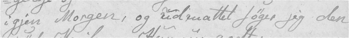igjen Morgen, og udmattet søger jeg den
